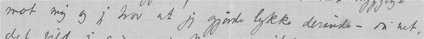mot mig og jeg tror at jeg gjorde lykke derinde – du vet,
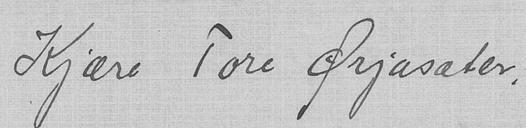Kjære Tore Ørjasæter,
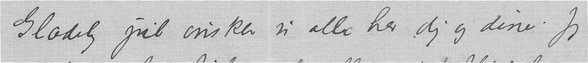Glædelig jul ønsker vi alle her dig og dine. Jeg
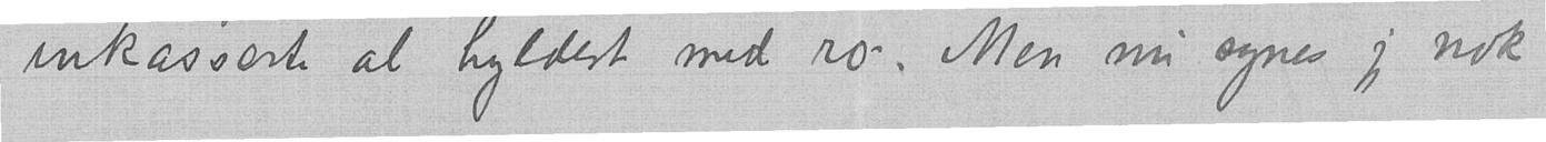inkasserte al hyldest med ro . Men nu synes jeg nok
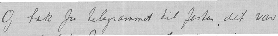Og tak for telegrammet til festen, det var
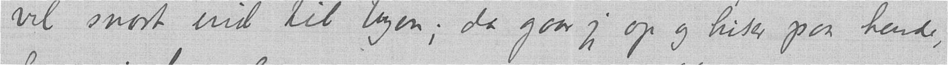vel snart ind til byen; da gaar jeg op og hilser paa hende,
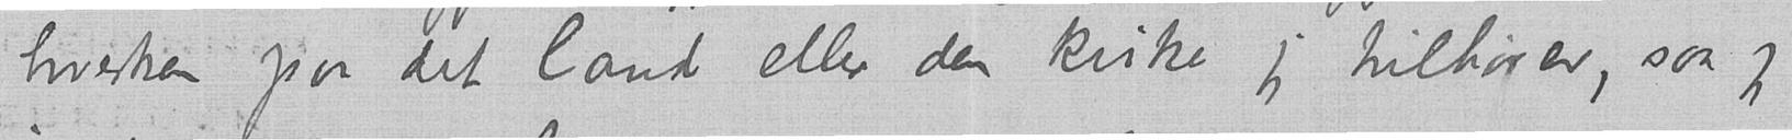hverken paa det land eller den kirke jeg tilhører, saa jeg
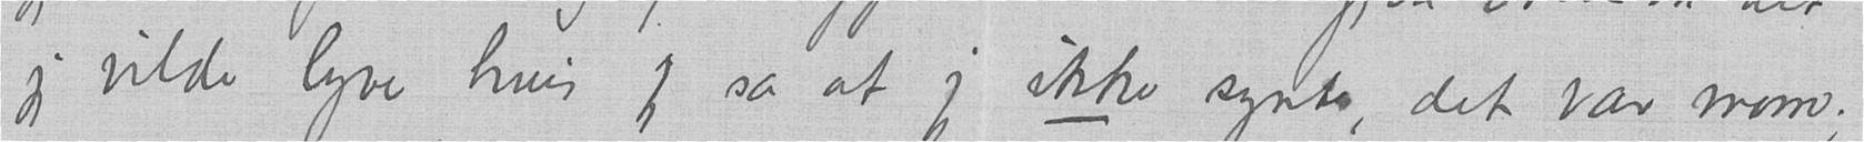jeg vilde lyve hvis jeg sa at jeg ikke syntes, det var morro;
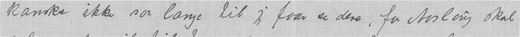kanske ikke saa længe til jeg faar se dere, for Aaslaug skal
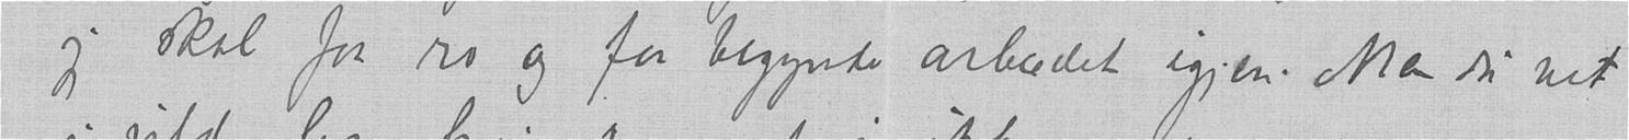jeg skal faa ro og faa begynde arbeidet igjen. Men du vet
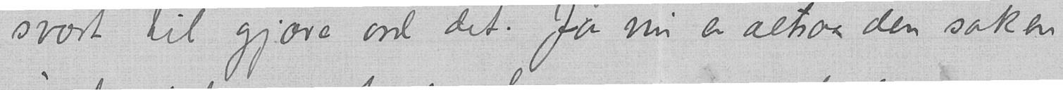svært til gjæve ord det. Ja nu er altsaa den saken
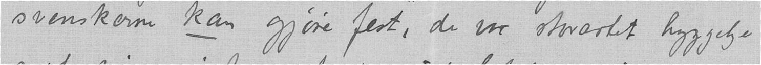svenskerne kan gjøre fest, de var storartet hyggelige
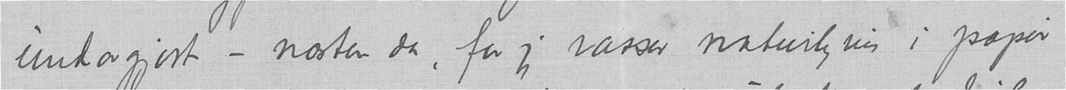undavgjort – næsten da, for jeg vasser naturligvis i papir
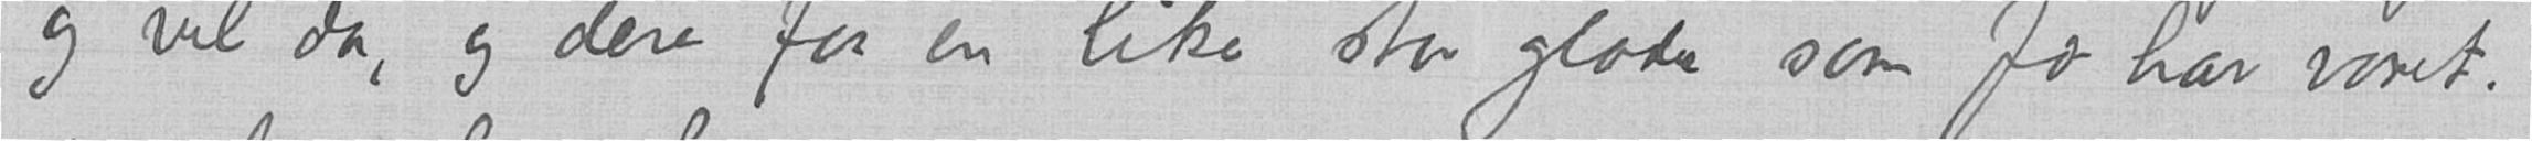og vel da, og dere faa en like stor glæde som Jo har været.
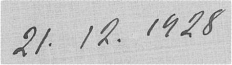21.12.1928
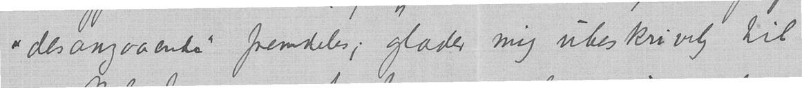"desangaaende" fremdeles; glæder mig ubeskrivelig til
